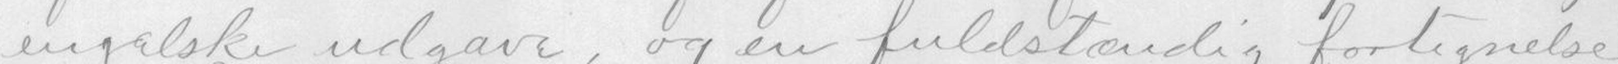engelske udgave, og en fuldstændig fortegnelse
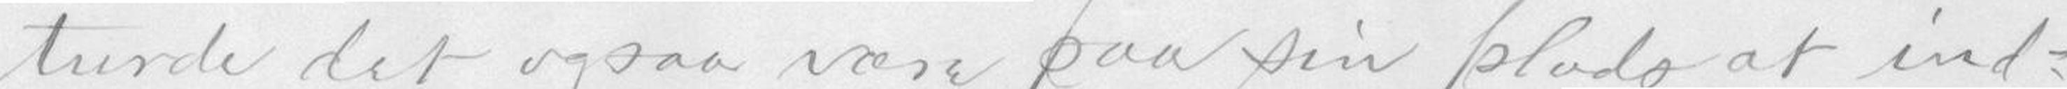turde det ogsaa være paa sin plads at ind-
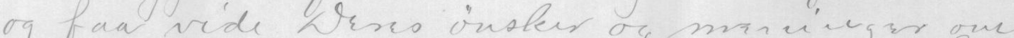og faa vide Deres ønsker og meninger om
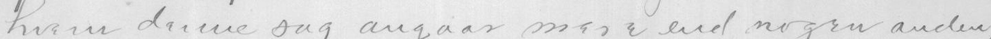hvem denne sag angaar mere end nogen andre,
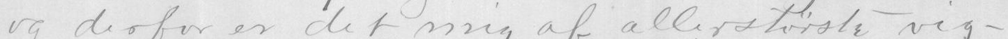og derfor er det mig af allerstørste vig-
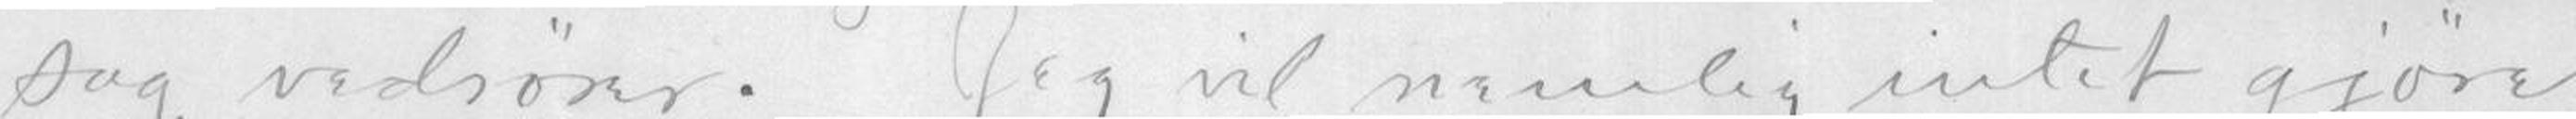sag vedrører. Jeg vil nemlig intet gjøre
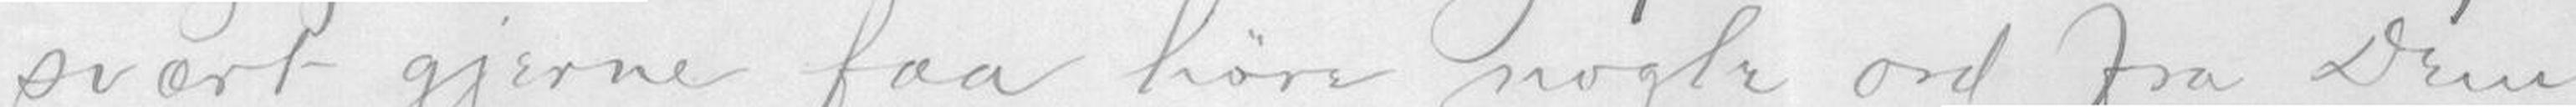svært gjerne faa høre nogle ord fra Dem
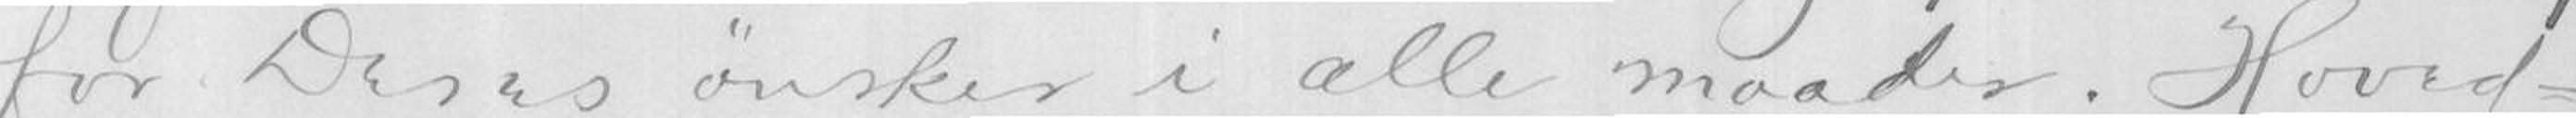for Deres ønsker i alle maader. Hoved-
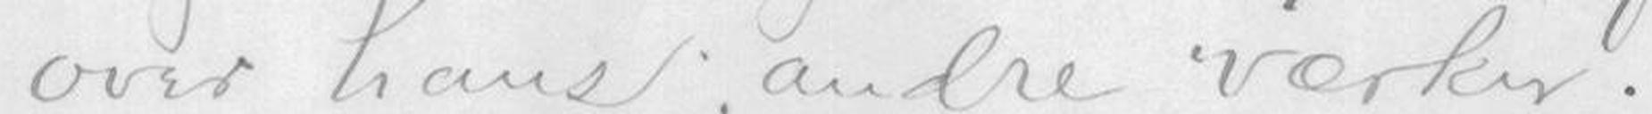over hans andre værke.
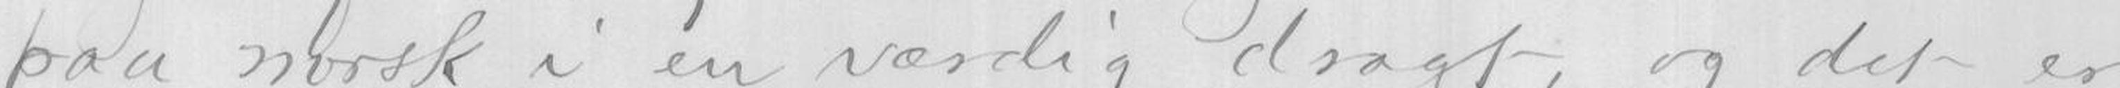paa norsk i en værdig dragt, og det er
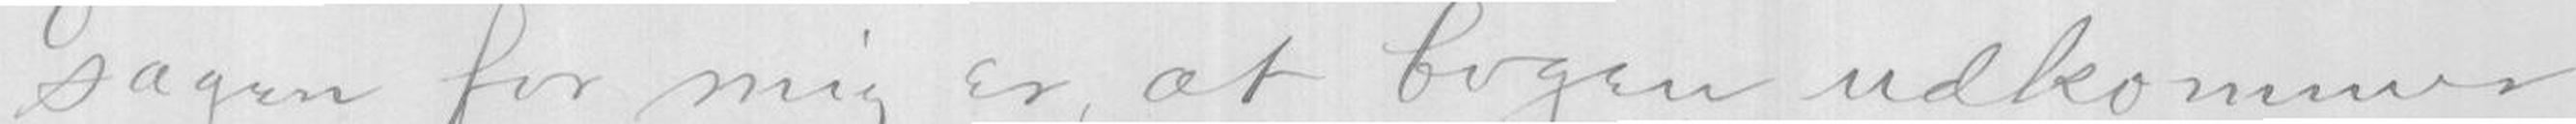sagen for mig er, at bogen udkommer
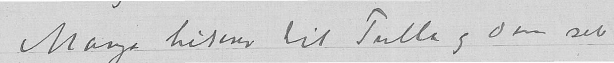Mange hilsener til Tulla og Dem selv
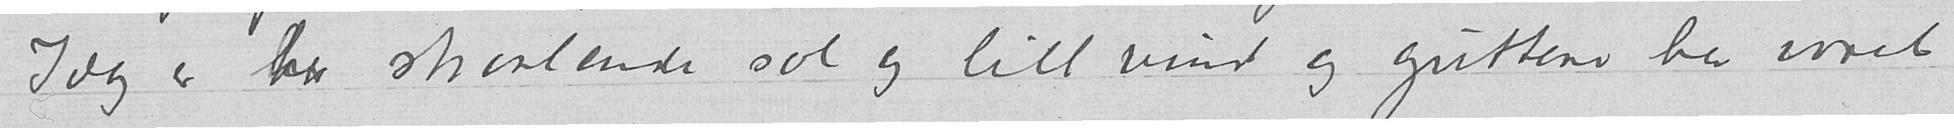Idag er her straalende sol og litt vind og guttene har været
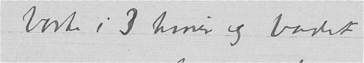borte i 3 timer og badet
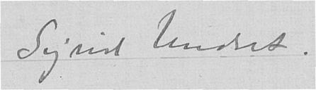Sigrid Undset.
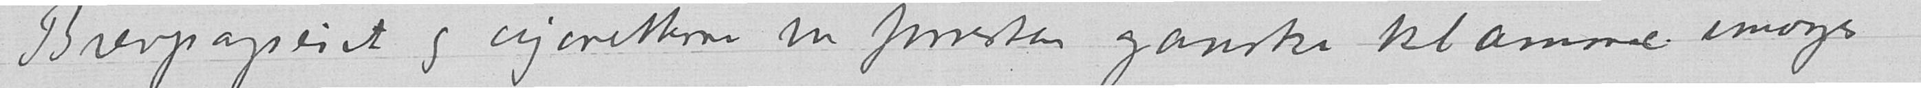Brevpapiret og cigaretterne var forresten ganske klamme imorges
